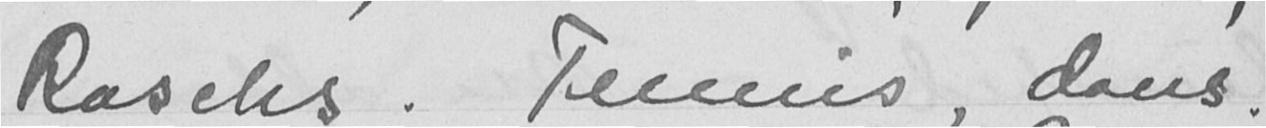Raschs. Tennis, dans.
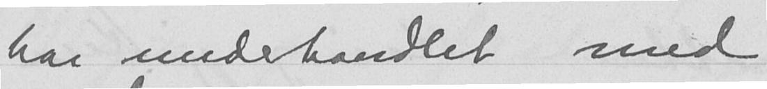har underhandlet med
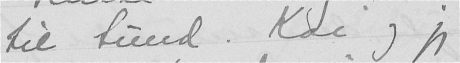til Sund. Kai og jeg
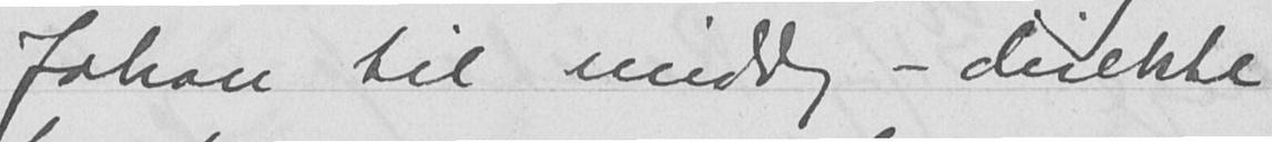Johan til middag - direkte
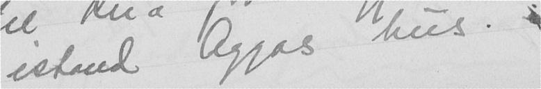istand Aggas hus. -
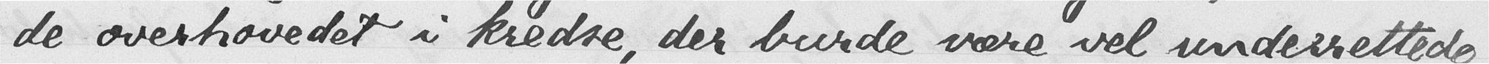de overhovedet i kredse, der burde være vel underrettede,
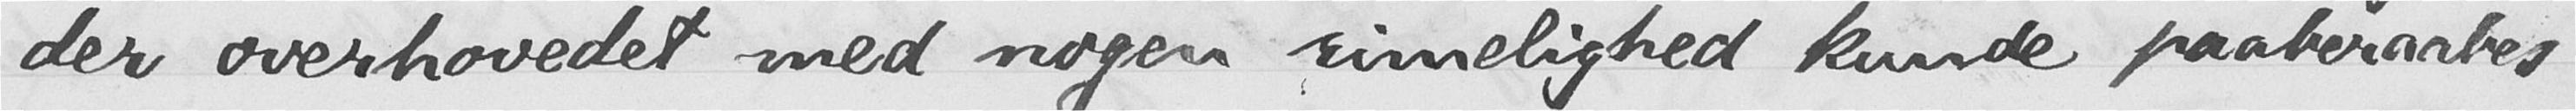der overhovedet med nogen rimelighed kunde paaberaabes
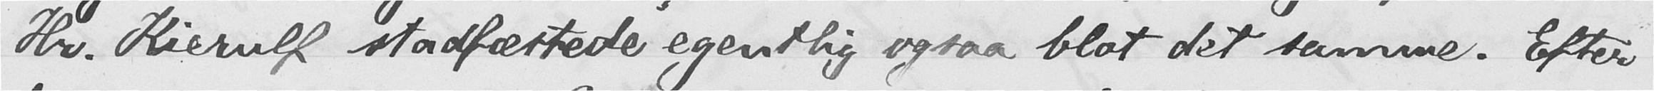Hr. Kierulf stadfæstede egentlig ogsaa blot det samme. Efter
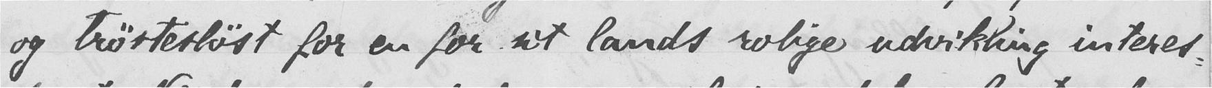og trøstesløst for en for sit lands rolige udvikling interes-
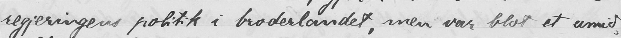regjeringens politik i broderlandet, men var blot et umid-
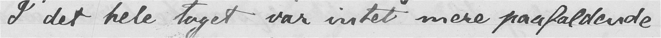I det hele taget var intet mere paafaldende
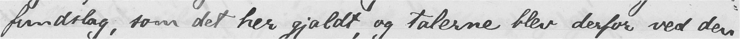fundslag, som det her gjaldt, og talerne blev derfor ved den
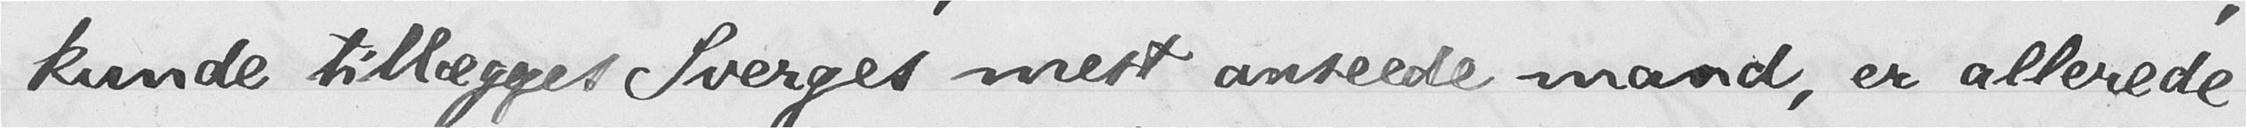kunde tillægges Sverges mest anseede mand, er allerede
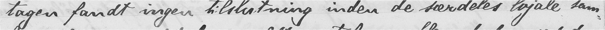tagen fandt ingen tilslutning inden de særdeles lojale sam-
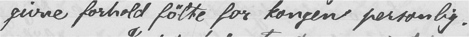givne forhold følte for kongen personlig.
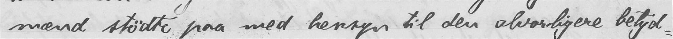mænd stødte paa med hensyn til den alvorligere betyd-
